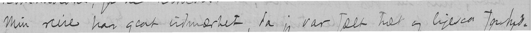Min reise har gaat udmærket, da jeg var fælt træt og ligesaa forhut-
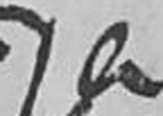ja
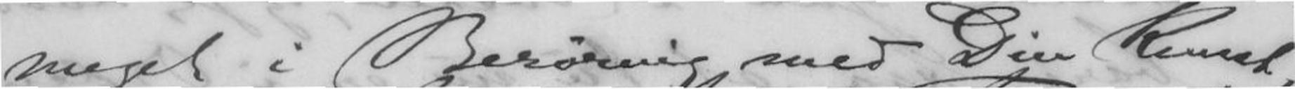meget i Berøring med Din Kunst,
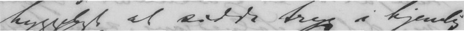hyggeligt at sidde tryg i hjemlig
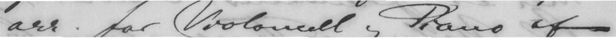arr. for Violoncell og Piano af
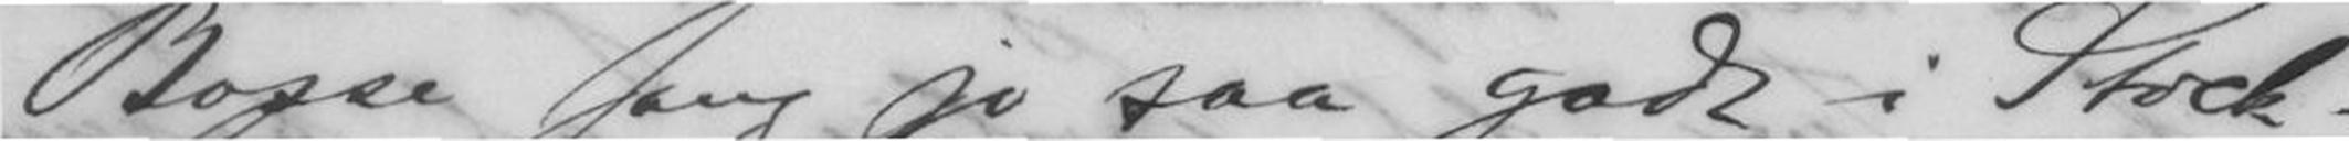Bosse sang jo saa godt i Stock-
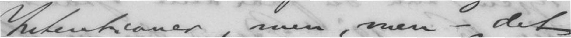Intentioner, men, men - det
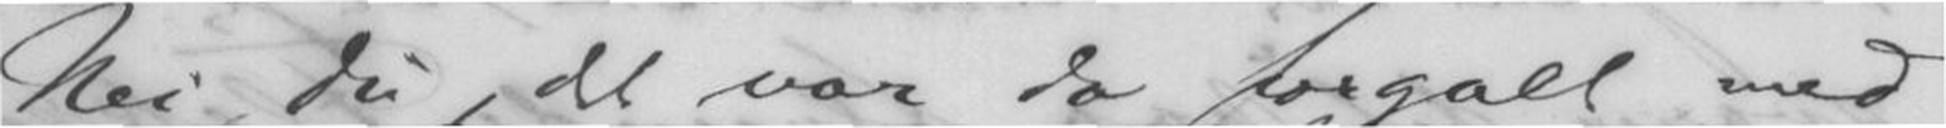Nei du det var da forgalt med
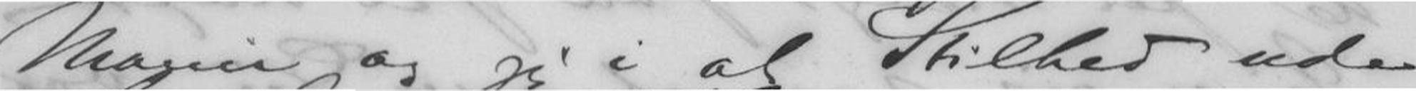Marie og jeg i al Stilhed ude
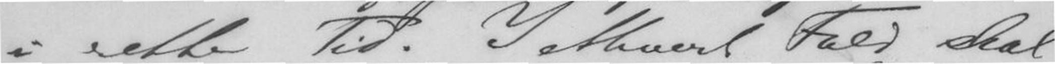i rette Tid. I ethvert Fald skal
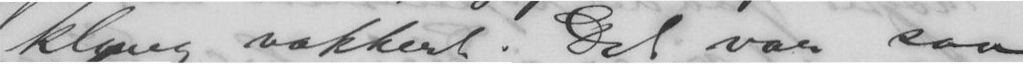klang vakkert. Det var saa
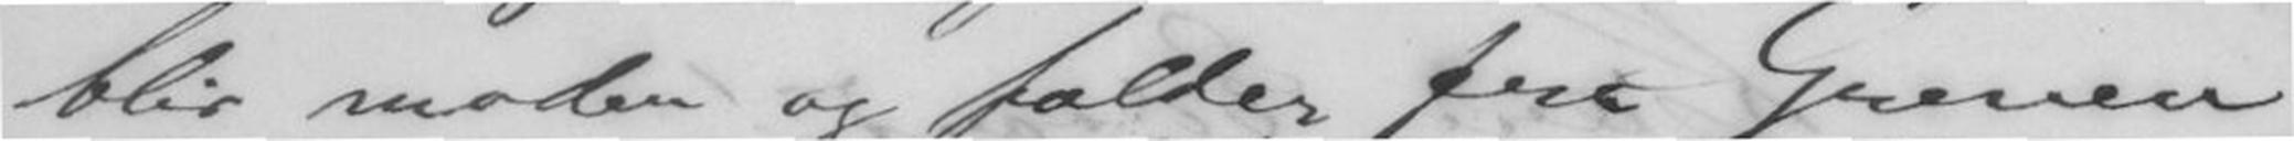blir moden og falder fra Grenen
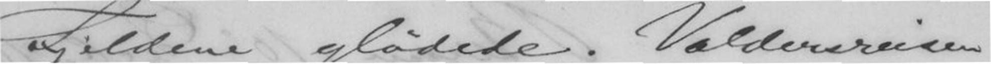Fjeldene glødede. Valdersreisen
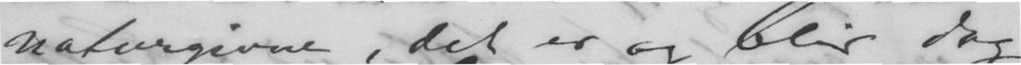naturgivne, det er og blir dog
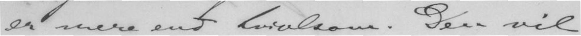er mere end tvivlsom. Den vil
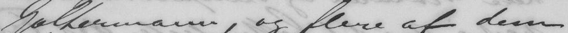Goltermann, og flere af dem
